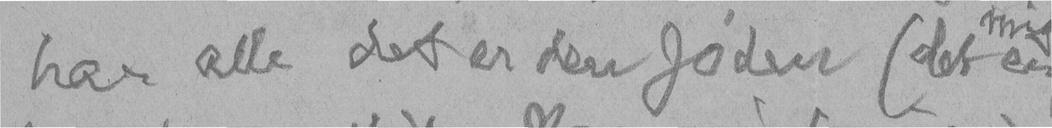har alle det er den Jøden (det er
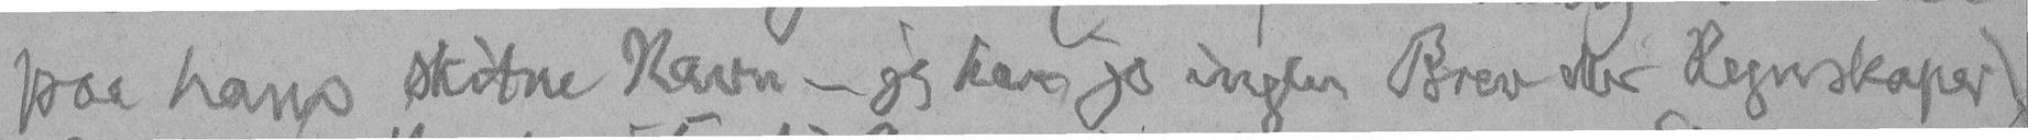paa hans skitne Navn - jeg har jo ingen Brev eller Regnskaper)
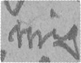mig
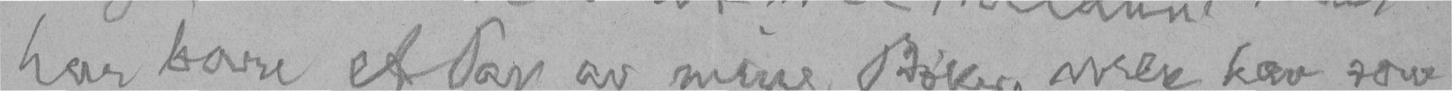har bare et Par av mine Bøker, men han som
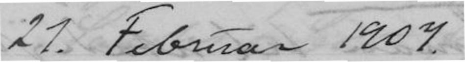21. Februar 1907.
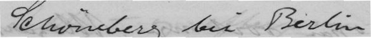Schöneberg bei Berlin
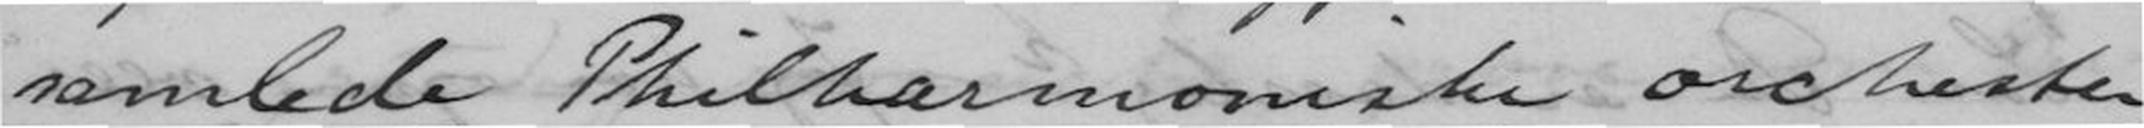samlede Philharmoniske orchester -
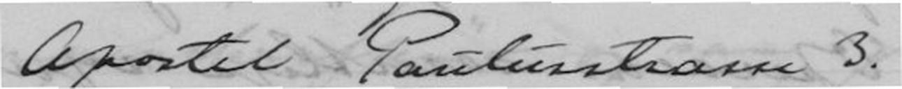Apostel Paulusstrasse 3.
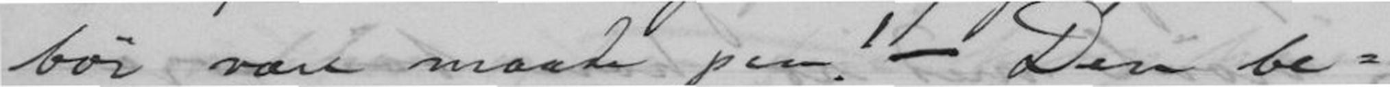bør være maade paa! - Den be-
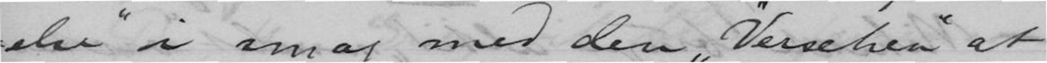else" i smag med den "Versehen" at
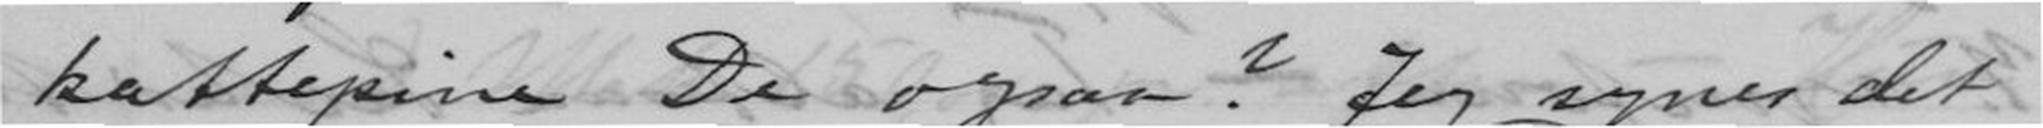kattepine De ogsaa? Jeg synes det
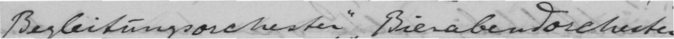"Begleitungsorchester", "Bierabendorchester",
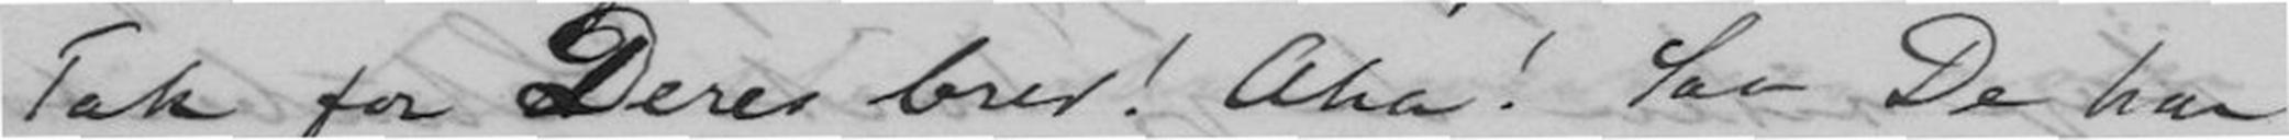Tak for Deres brev! Aha! Saa De har
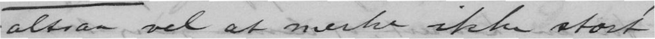altsaa vel at merke ikke stort
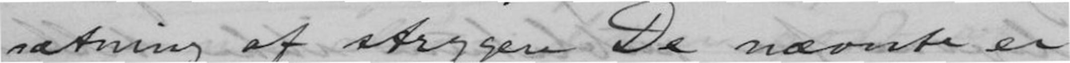ætning af strygere De nævnte er
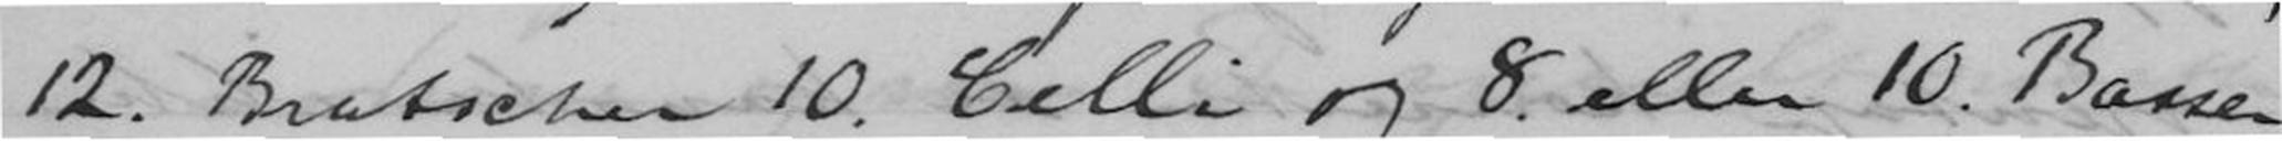12. Bratscher 10. Celli og 8. eller 10. Basser.
